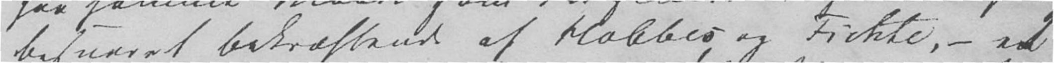besvaret bekræftende af Hobbes og Fichte, – et
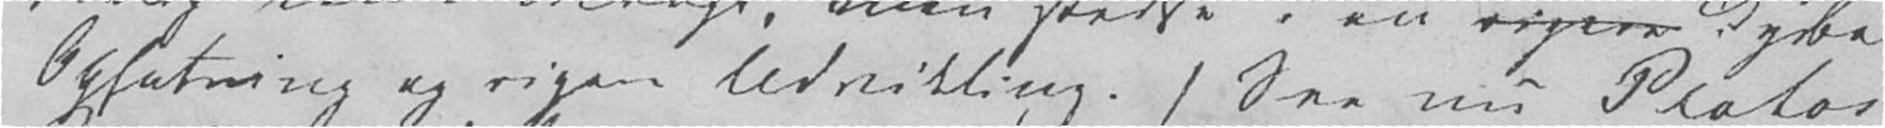Opfatning og rigere Udvikling. (See nu Platos
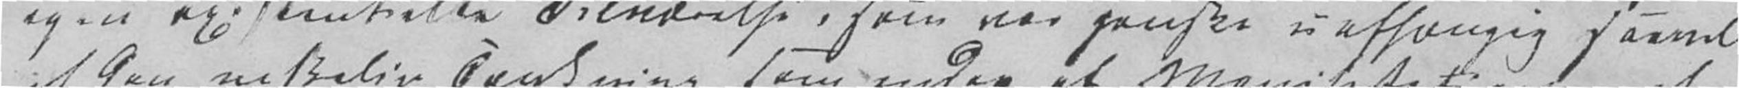egen existentielle Tilværelse, som var ganske uafhængig saavel
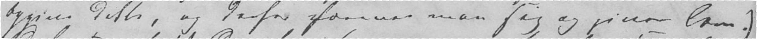opgive dette, og derfor forener man sig og giver Love.)
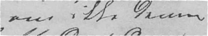om ikke denne
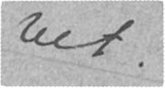vet.
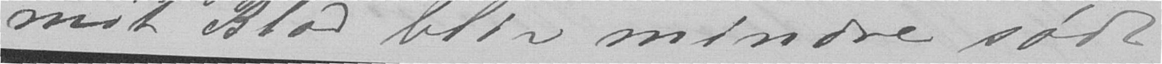mit Blod blir mindre sødt
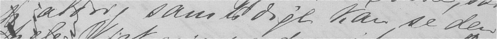jeg aldrig samtidigt kan se den
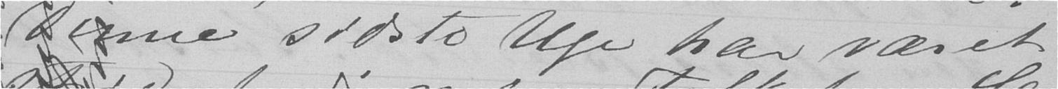Denne sidste Uge har været
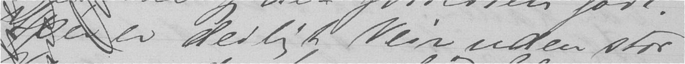Her er deiligt Veir uden stor
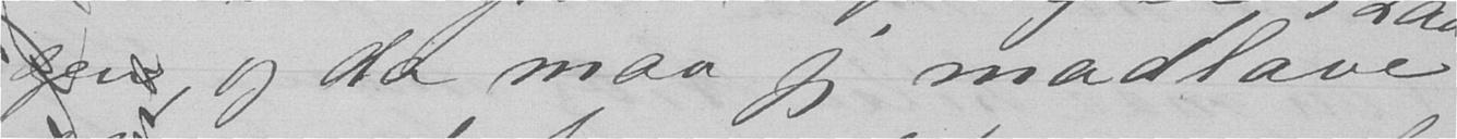gen, og da maa jeg madlave
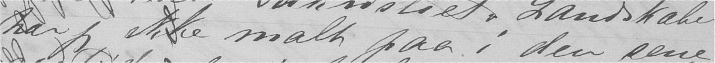har jeg ikke malt paa i den sene-
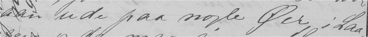leleir ude paa nogle Øer i Laa-
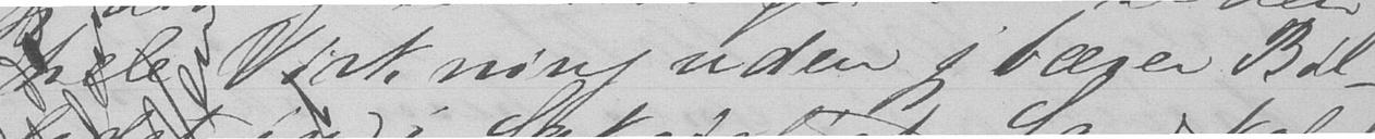hele Virkning uden jeg bærer Bil-
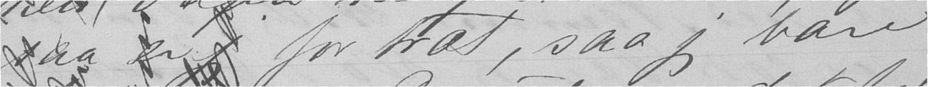saa er jeg for træt, saa jeg bare
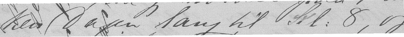ken Dagen lang til Kl. 8, og
